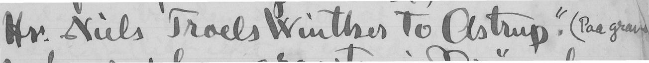"Hr. Niels Troels Winther to Astrup", (Paa graven
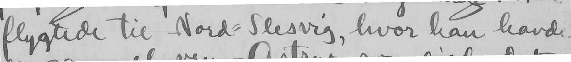flygtede til Nord-Slesvig, hvor han havde
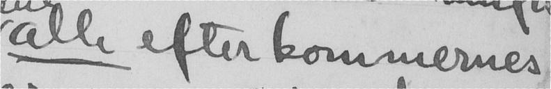alle efter kommernes
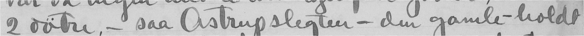2 døtre, - saa Astrupslegten - den gamle - holdt
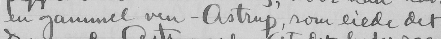en gammel ven - Astrup, som eiede det
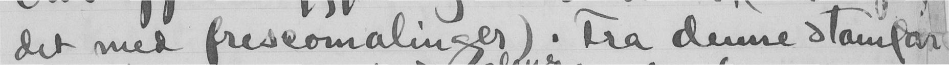det med frescomalinger). dra denne stamfar
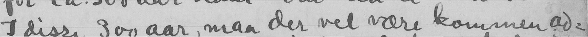I disse 300 aar maa der vel være kommen ad-
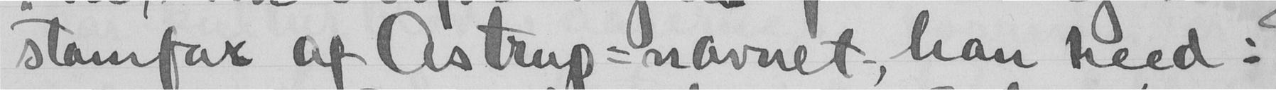stamfar af Astrup-navnet, han heed:
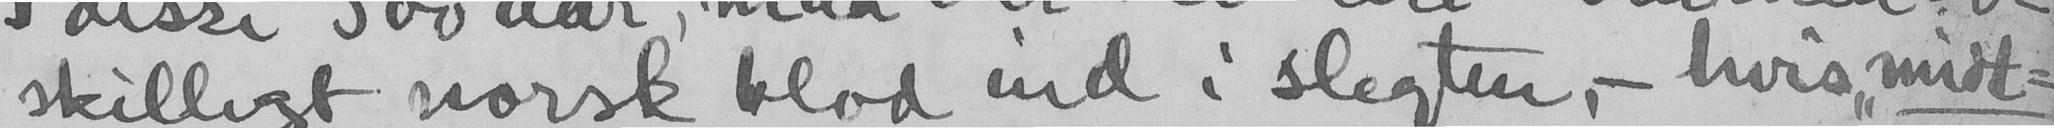skilligt norsk blod ind i slegten, - hvis "midt-
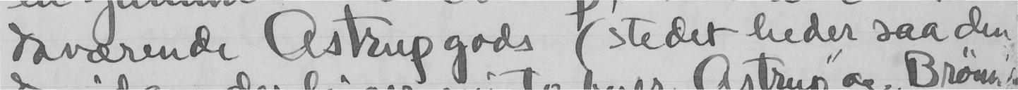daværende Astrupgods (stedet heder saa den
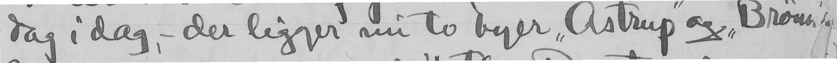dag i dag, - der ligger nu to byer "Astrup" og "Brønh";
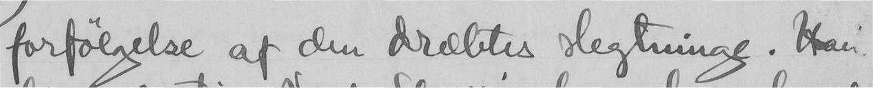forfølgelse af den drebtes slegtninge. Han
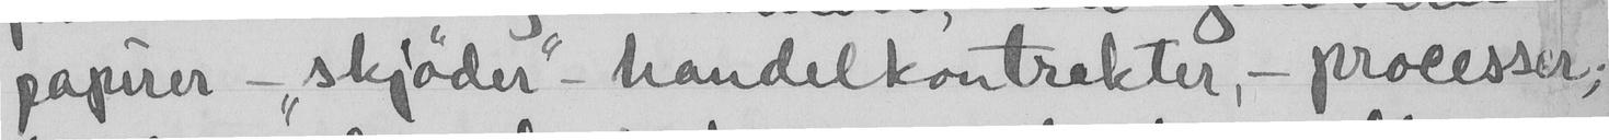papirer - "skjøder" - handelkontrakter, - processer;
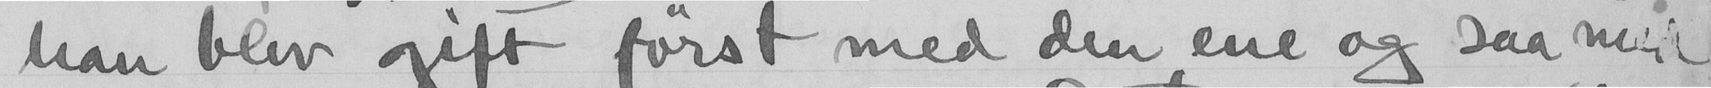han blev gift først med den ene og saa med
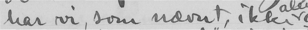har vi, som nævnt, ikke
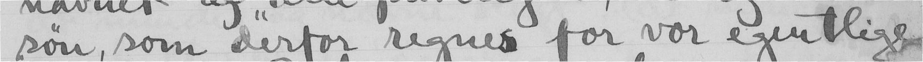søn, som derfor regnes for vor egentligee
